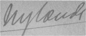Nylænde
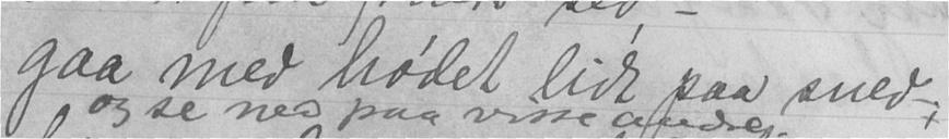gaa med hodet lidt paa sned-;
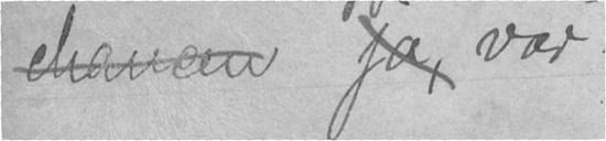chancen ja, var
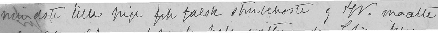mindste lille pige fik falsk strubehoste og W. maatte
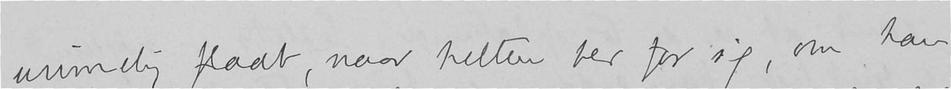urimelig fladt, naar helten ber for sig, om han
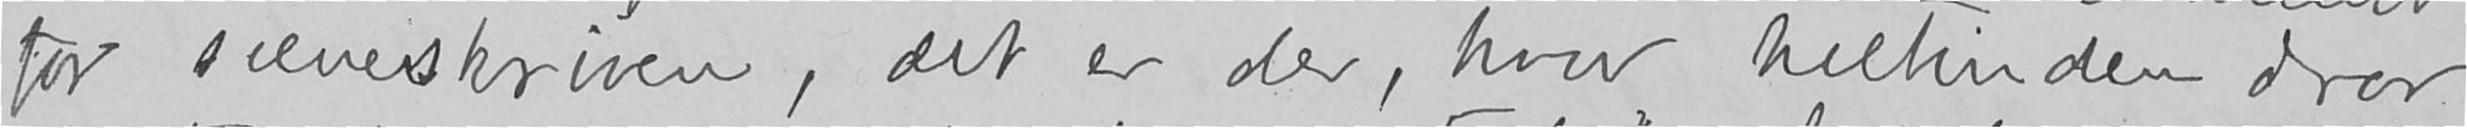for sceneskriven, det er der, hvor heltinden drar
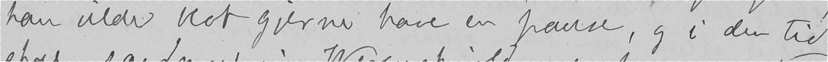han vilde blot gjerne have en pause, og i den tid
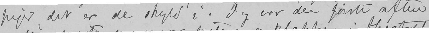piger, det er de skyld i. Jeg var der første aften,
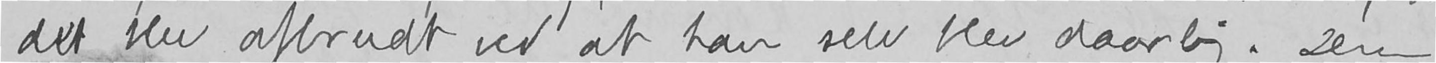det blev afbrudt ved at han selv blev daarlig. Deres
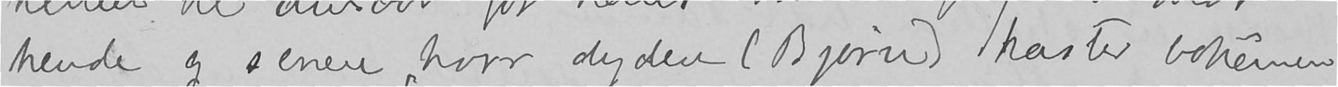hende og senere, hvor dyden (Bjørn) kaster bohémen
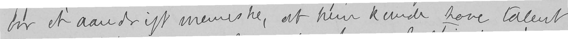var et aandrigt menneske, at hun kunde have talent
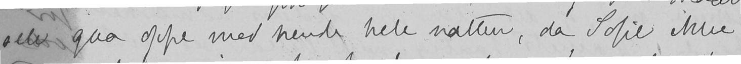selv gaa oppe med hende hele natten, da Sofie ikke
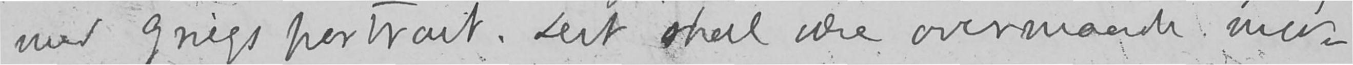med Griegs portrait. Det skal være overmaade mor-
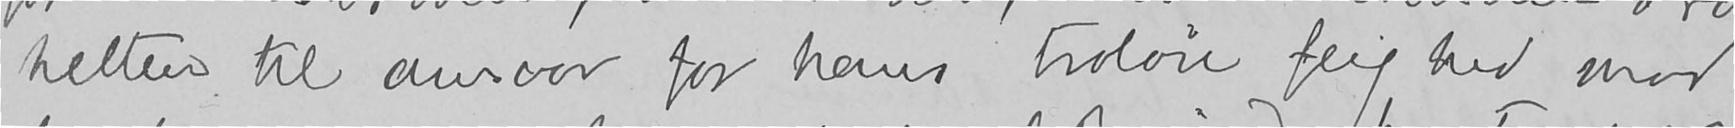helten til ansvar for hans troløse feighed mod
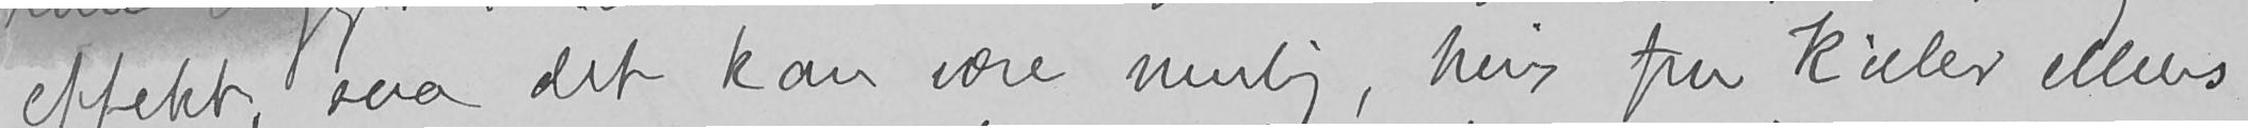effekt, saa det kan være mulig, hvis fru Kieler ellers
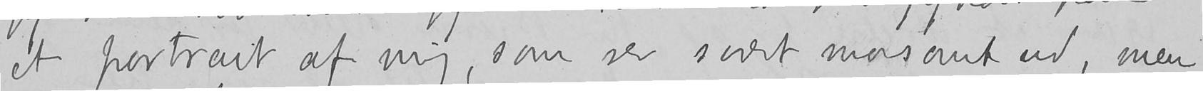et portrait af mig, som ser svært morsomt ud, men
